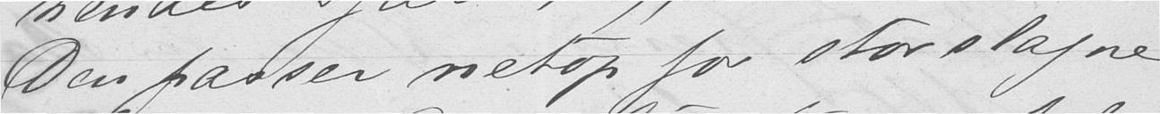Den passer netop for storslagne
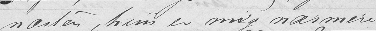næsten, hun er mig nærmere
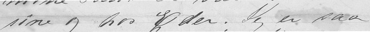sine og hos Eder. Jeg er saa
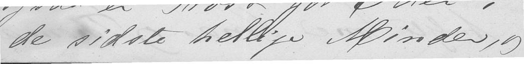de sidste hellige Minder, og
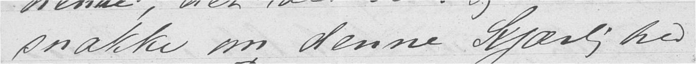snakke om denne Kjærlighed
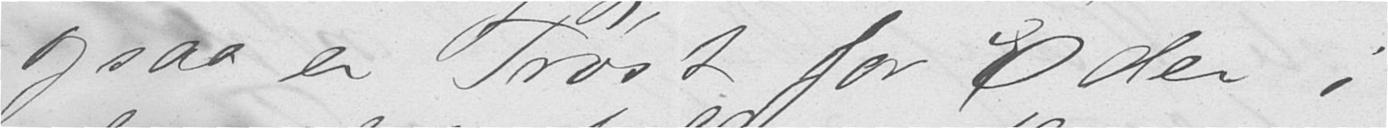ogsaa er Trøst for Eder i
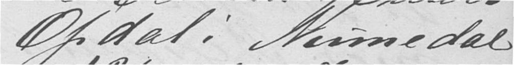Opdal i Numedal
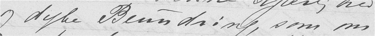og dybe Beundring, som om
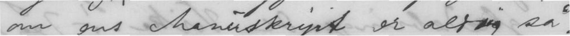om ens Manuskript er aldrig så
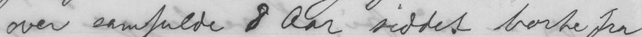over samfulde 8 Aar siddet borte fra
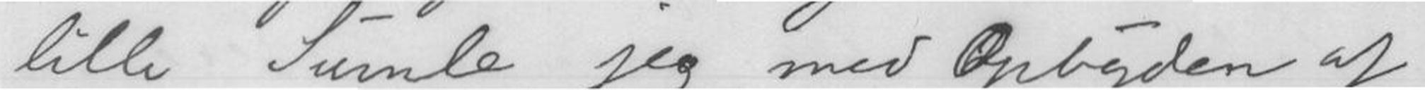lille Smule jeg med Opbyden af
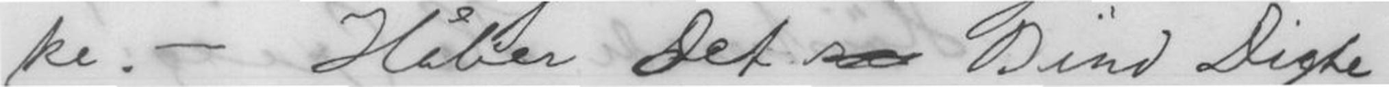ke. - Håber Det Bind Digte
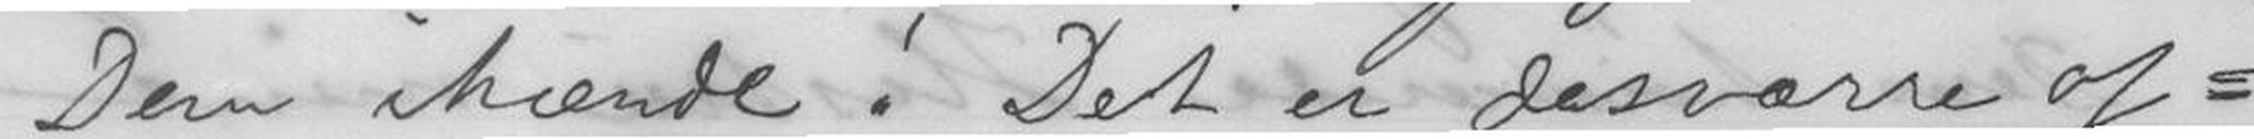Dem ihænde! Det er desværre of-
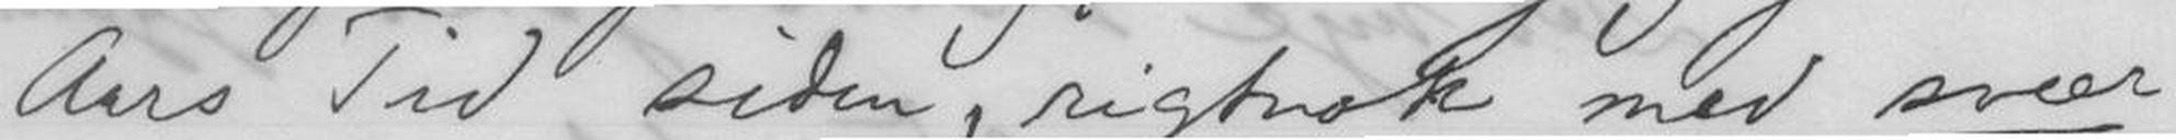Aars Tid siden, rigtnok med svær
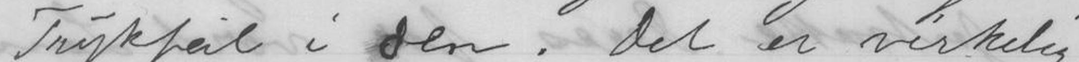Trykfeil i Den. Det er virkelig
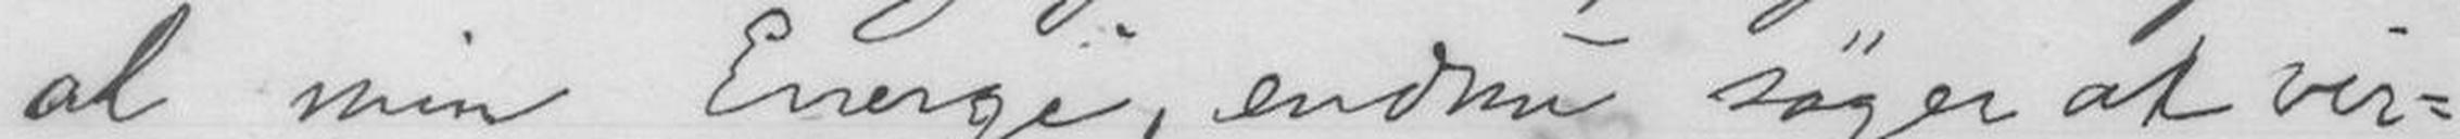al min Energi, endnu søger at vir-
